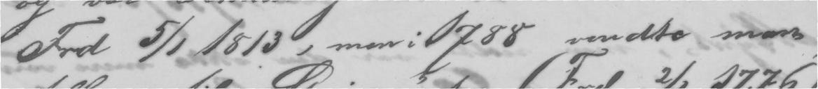Frd 5/1 1813, men i 1788 vendte man
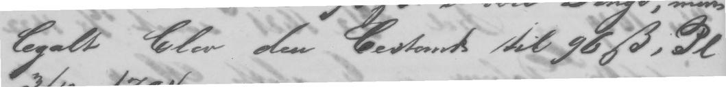legalt blev den bestemt til 96 ẞ, Pl
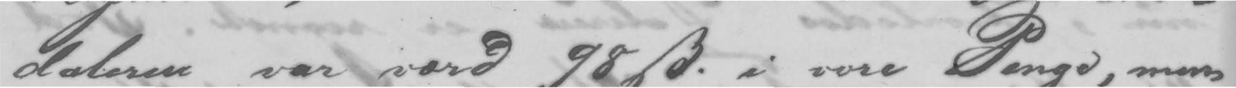daleren var værd 98 ẞ. i vore Penge, men
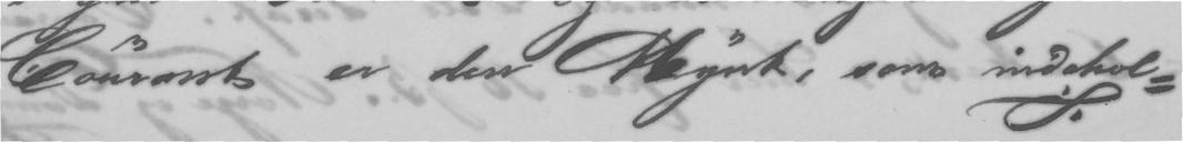Courant er den Mynt, som indehol-
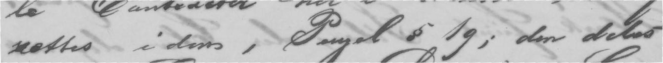sættes i den, Pengel § 19; i den deles
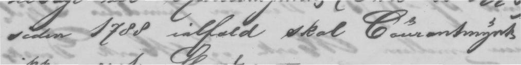siden 1788 ialfald skal Courantmynt
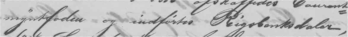myntfoden og indførtes Rigsbanksdaler,
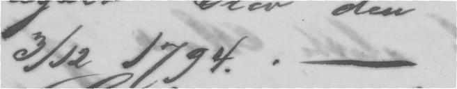3/12 1794. -
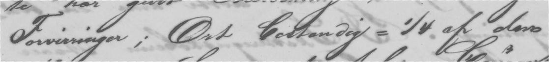Forvirringer; Ort bestandig = ¼ af den
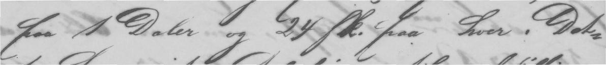paa 1 Daler og 24 fk. paa hver. Det-
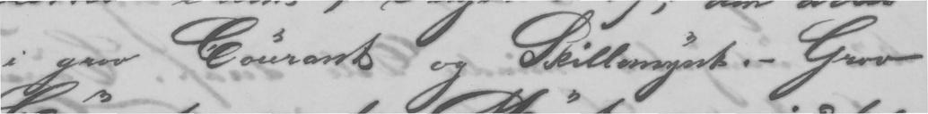i grov Courant og Skillemynt. - Grov
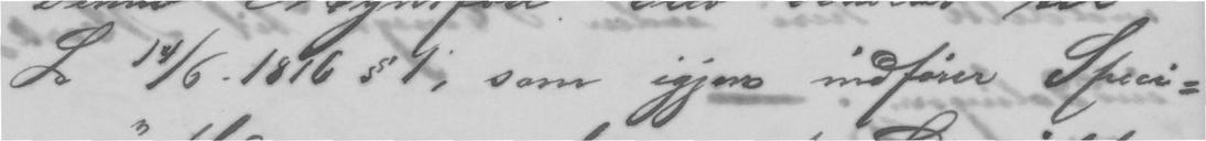L 14/6-1816 §1, som igjen indfører Speci-
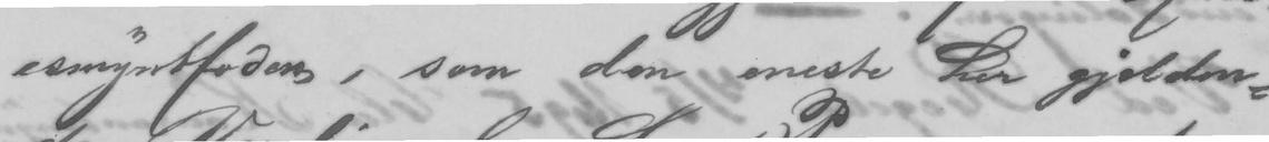esmyntfoden, som den eneste her gjelden-
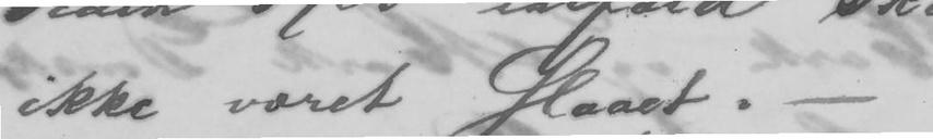ikke været slaaet. -
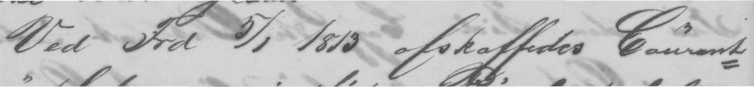Ved Frd 5/1 1813 afskaffedes Courant-
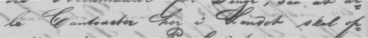le Contracter her i Landet skal op-
